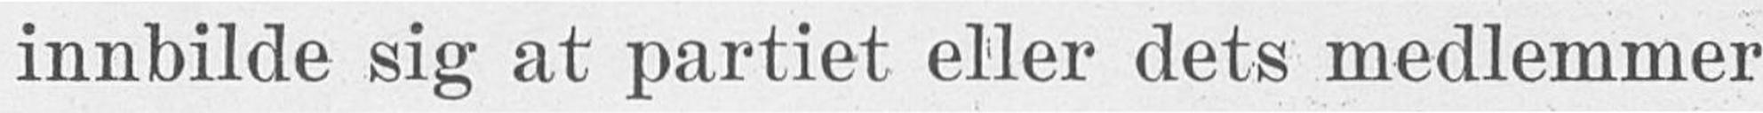innbilde sig at partiet eller dets medlemmer
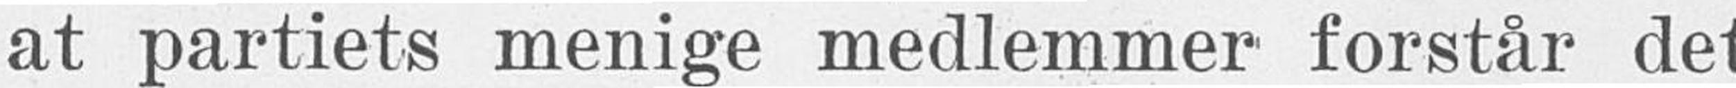at partiets menige medlemmer forstår det
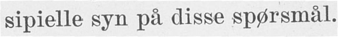sipielle syn på disse spørsmål.
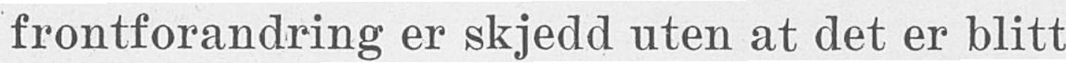frontforandring er skjedd uten at det er blitt
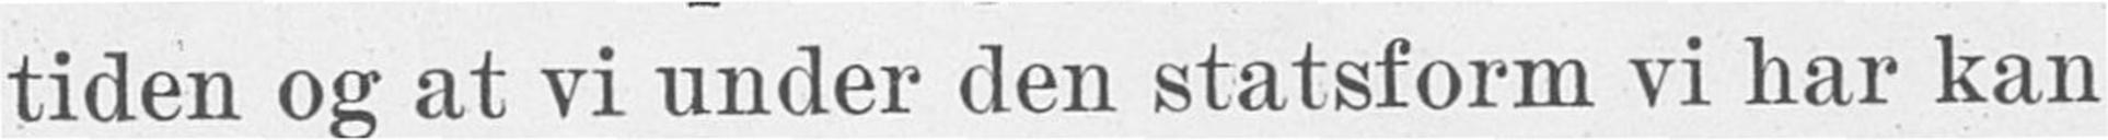tiden og at vi under den statsform vi har kan
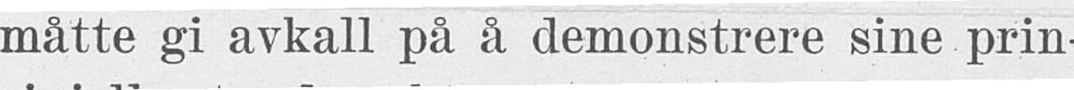måtte gi avkall på å demonstrere sine prin-
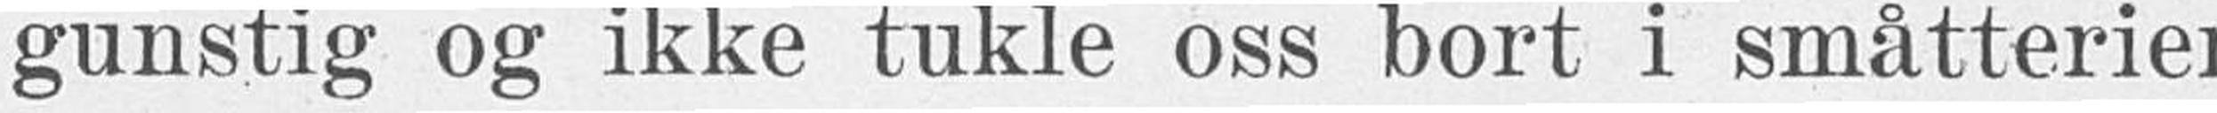gunstig og ikke tukle oss bort i småtteriei
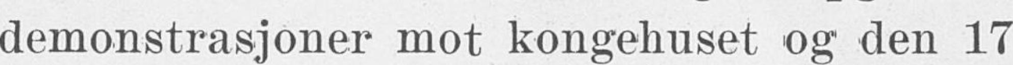demonstrasjoner mot kongehuset og den 17
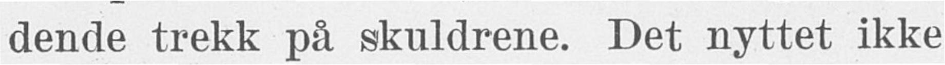dende trekk på skuldrene. Det nyttet ikke
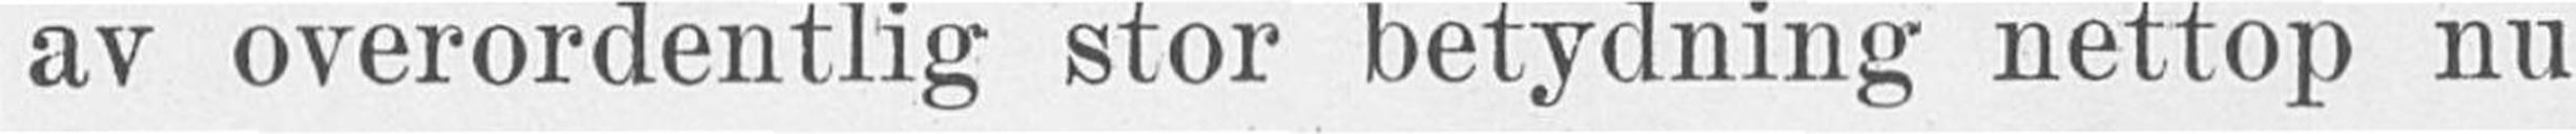av overordentlig stor betydning nettop nu
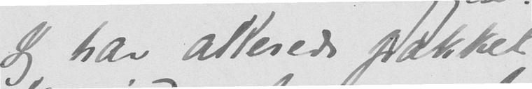Jeg har allerede pakket
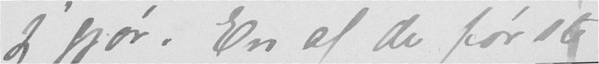jeg gjør. En af de første
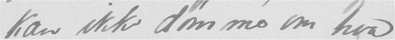kan ikke dømme om hvad
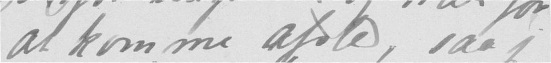at komme afsted, saa jeg
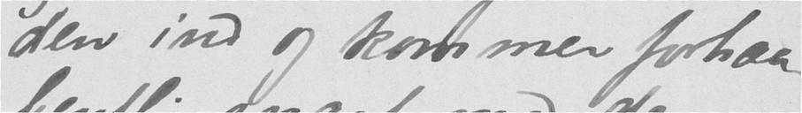den ind og kommer forhaa-
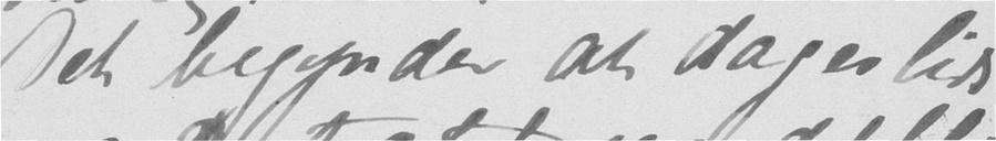Det begynder at dages lidt
